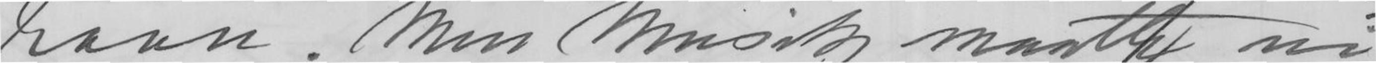havn. Men Musik maatte vi
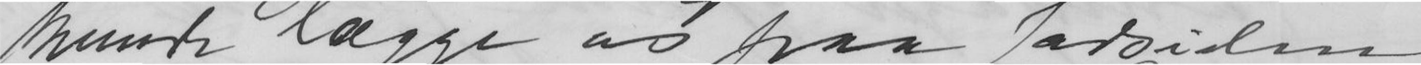kunde lægge os paa Ladsiden
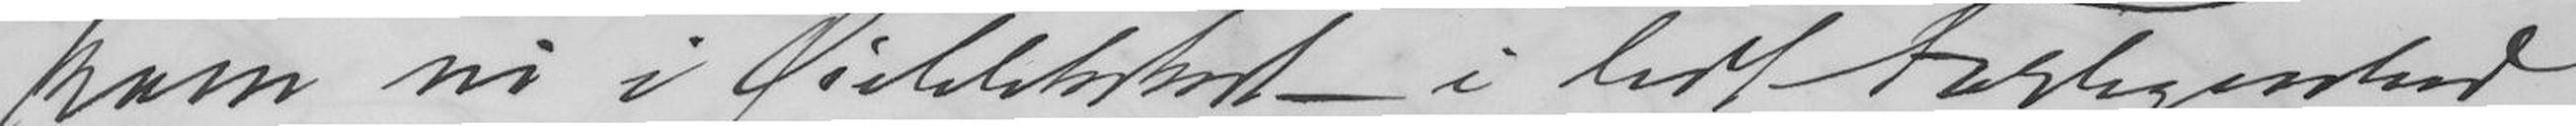kom vi i Øieblikket i lidt Forlegenhed
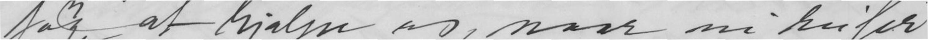søge at hjælpe os, naar vi reiser
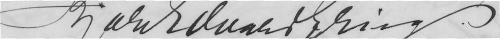Kjære Edvard Grieg!
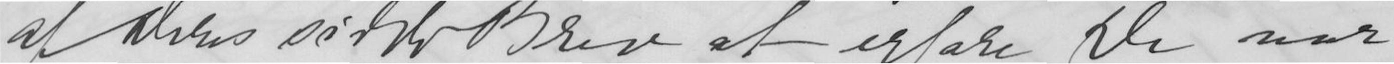af Deres sidste Brev at erfare De var
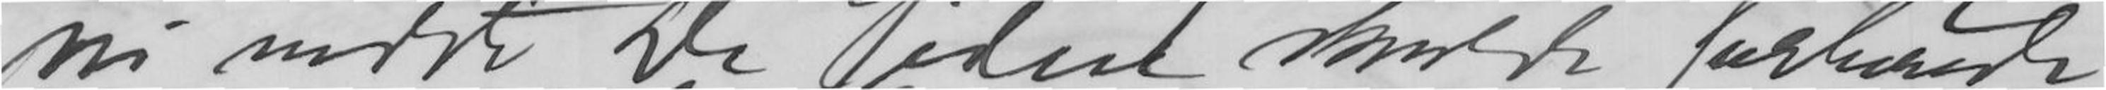vi vidste De siden skulde forberede
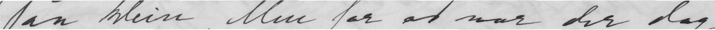saa klein. Men for os var der dog
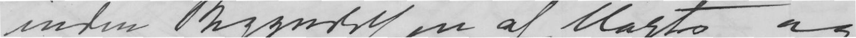inden Begyndelsen af Marts og
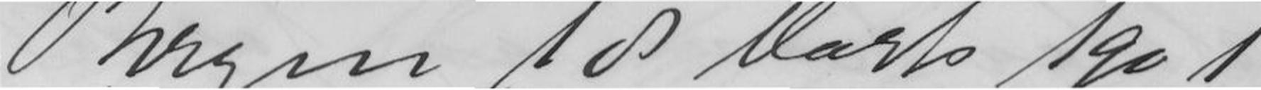Bergen 18 Marts 1901
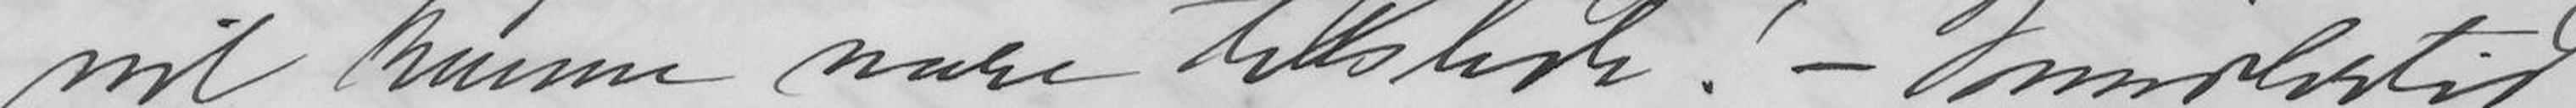vil kunne være tilstede! - Imidlertid
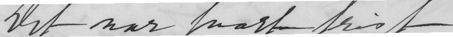Det var svært trist
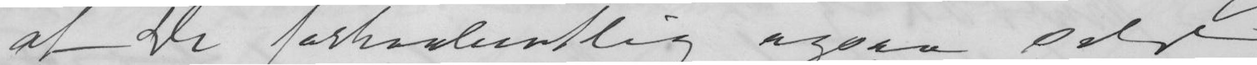at De forhaabentlig ogsaa selv
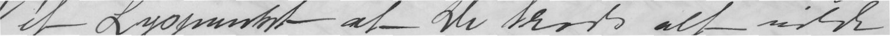ét Lyspunkt, at De trods alt vilde
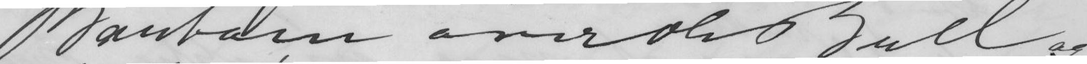Bautaen over Ole Bull og
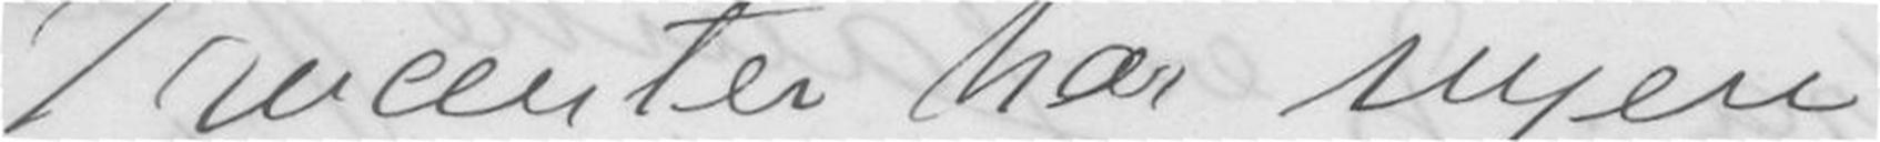Trocenter har ingen
